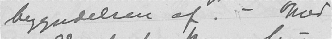begyndelsen af. - Med
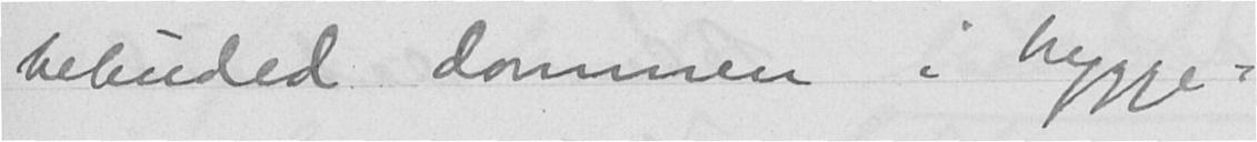bebuded dommen i brygge-
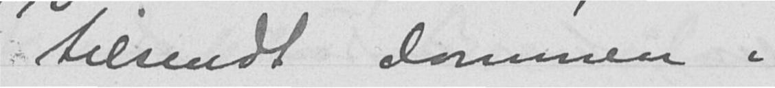tilsendt dommen i
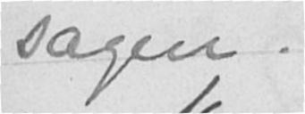sagen.
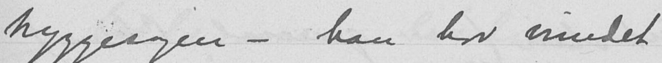bryggesagen - han har vundet
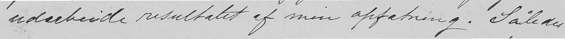udarbeide resultatet af min opfatning. Således
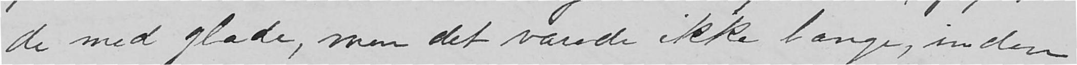de med glæde, men det varede ikke længe, inden
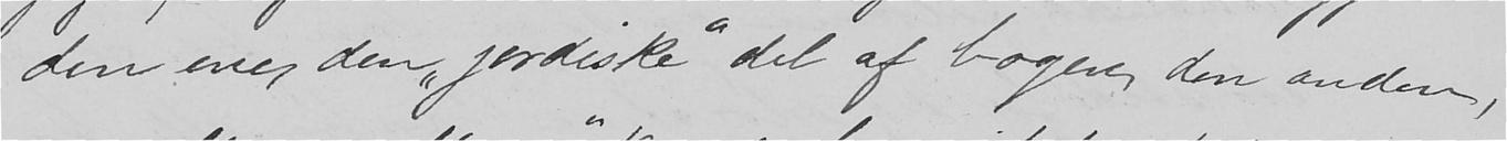den ene, den "jordiske" del af bogen den anden,
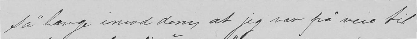så længe imod dem, at jeg var på veie til
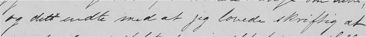og det endte med at jeg lovede skriftlig at
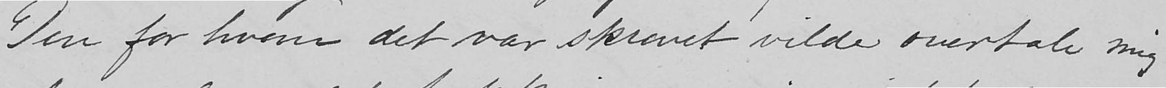Den for hvem det var skrevet vilde overtale mig
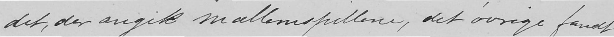det, der angik mællemspillene, det øvrige fandt
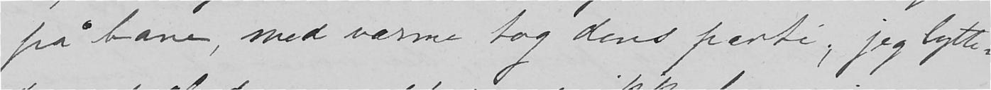på bane, med varme tog dens parti, jeg lytte-
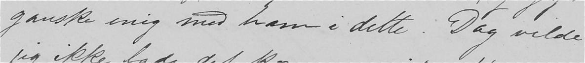ganske enig med ham i dette. Dog vilde
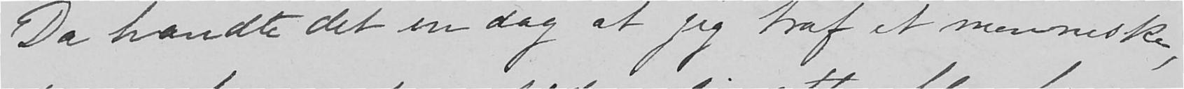Da hændte det en dag at jeg traf et menneske,
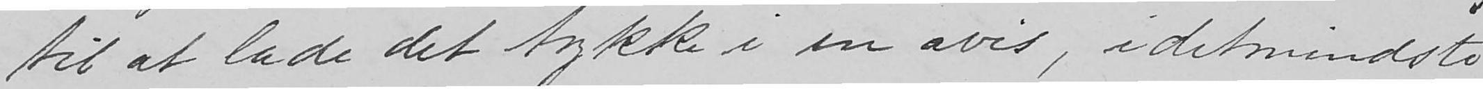til at lade det trykke i en avis, i detmindste
